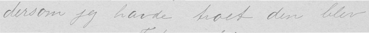dersom jeg havde troet den blev
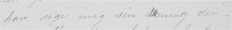kan sige mig sin Mening der-
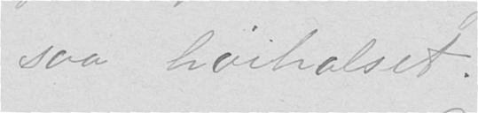saa høihalset.
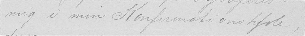mig i min Konfirmationskjole,
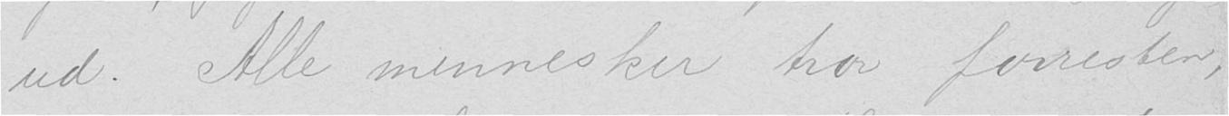ud. Alle mennesker tror forresten,
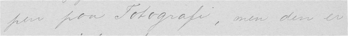pen paa Fotografi, men den er
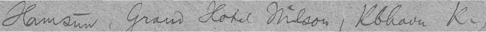Hamsun, Grand Hotel Nilson, Kbhavn K.
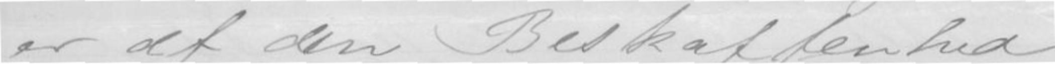er af den Beskaffenhed
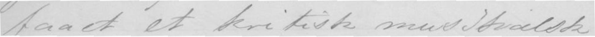faaet et kritisk musikalsk
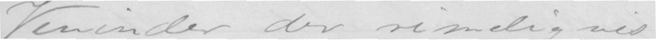Veninder der rimeligvis
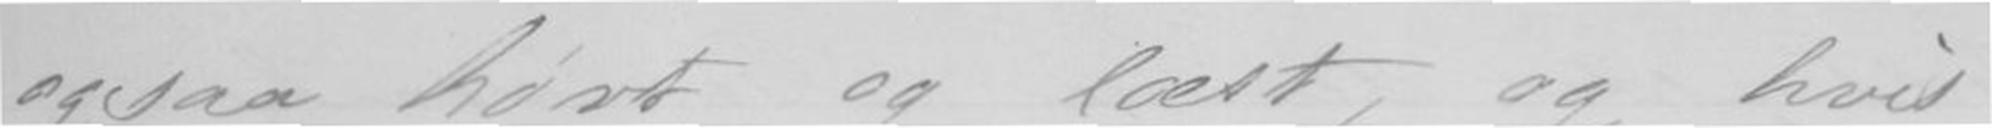ogsaa hørt og læst, og hvis
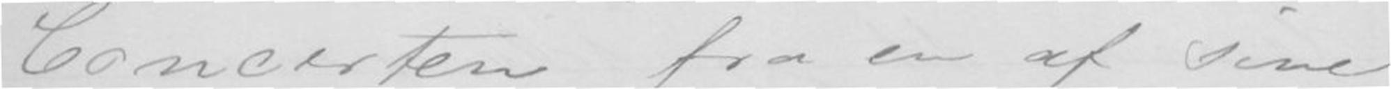Concerten fra en af sine
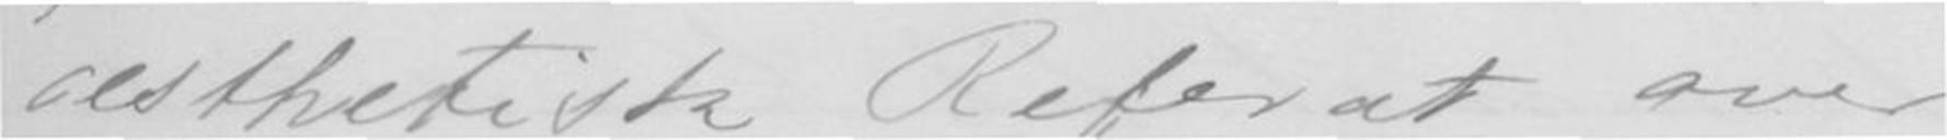alsthetisk Referat over
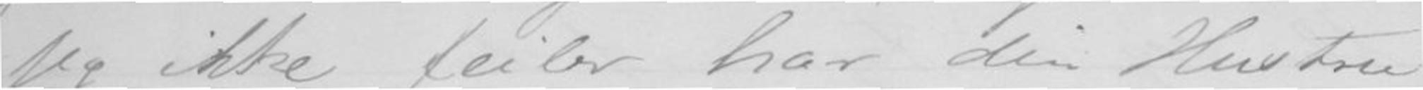jeg ikke feiler har din Hustru
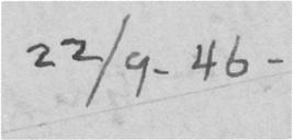22/9. 46.
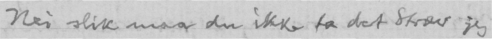Nei slik maa du ikke ta det Stræv jeg
